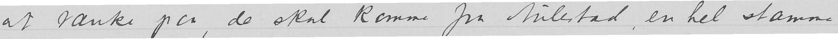at tænke paa, de skal komme fra Aulestad, en hel stamme
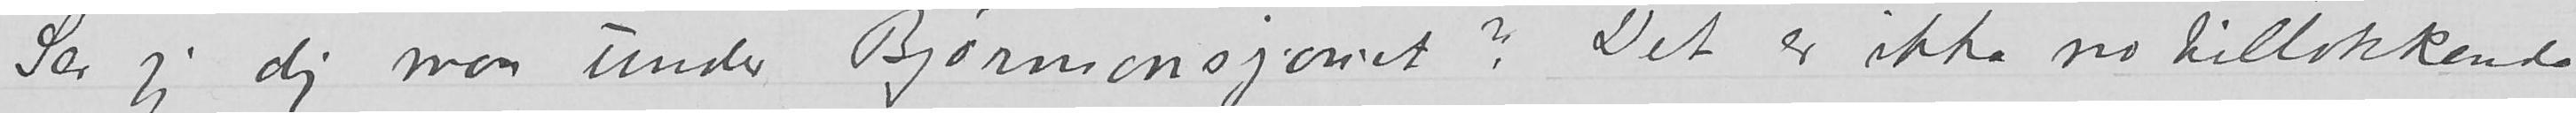Ser jeg dig mon under Bjørnsonsjauet? Det er ikke no tillokkends
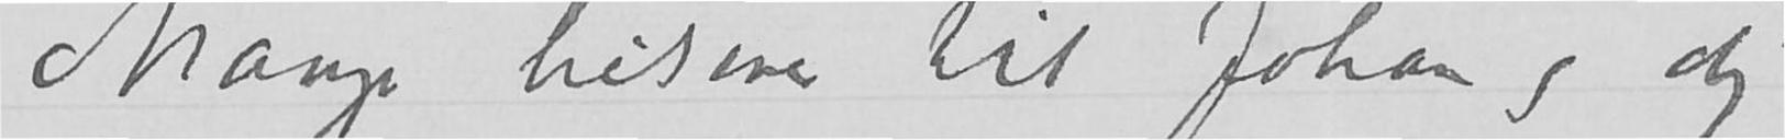Mange hilsener til Johan og dig
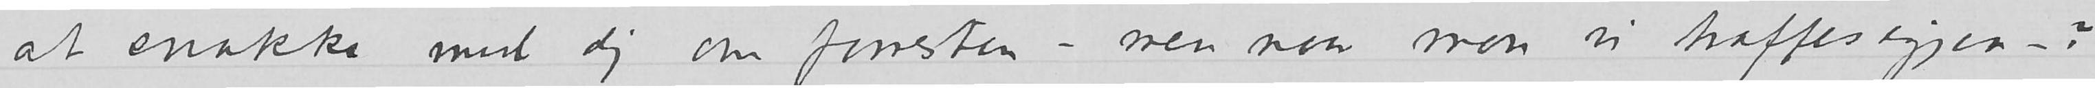at snakke med dig om forresten – men naar mon vi træffes igjen –?
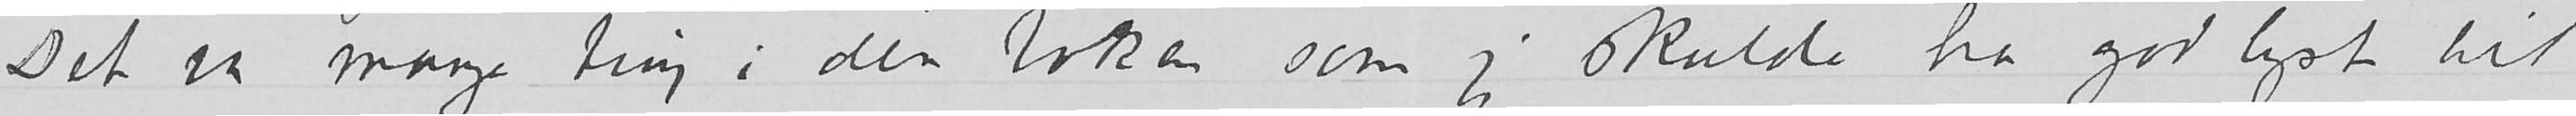Det var mange ting i den boken som jeg skulde ha god lyst til
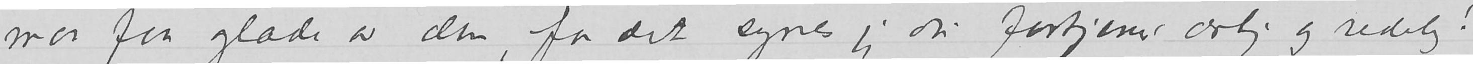du maa faa glæde av den, for det synes jeg du fortjener ærlig og redelig!
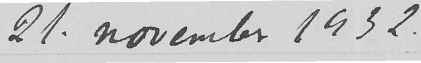21. november 1932.
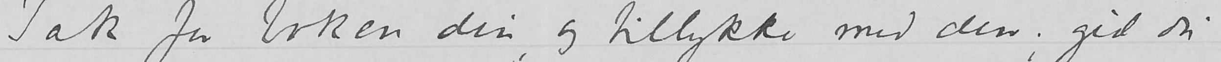Tak for boken din, og til lykke med den; gid
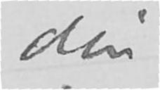din
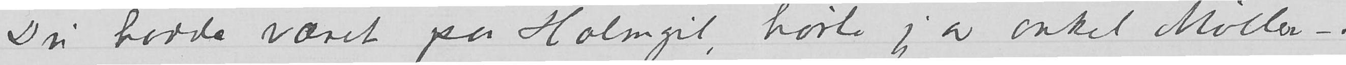Du hadde været paa Holmgil, hørte jeg av onkel Møller –.
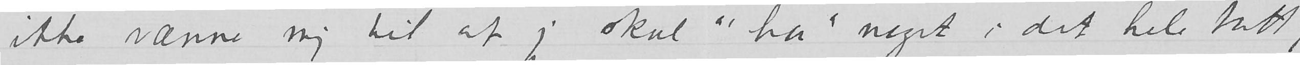ikke vænne mig til at jeg skal «ha» noget i det hele tatt;
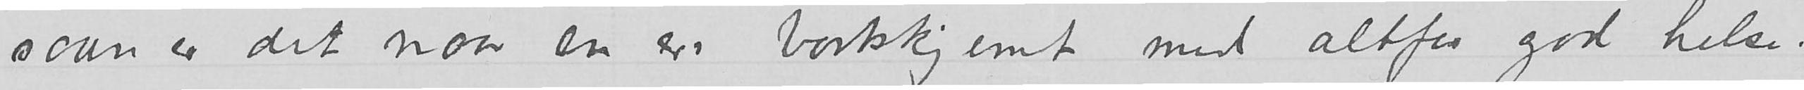saan er det naar en er bortskjemt med altfor god helse.
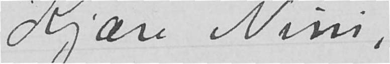Kjære Nini,
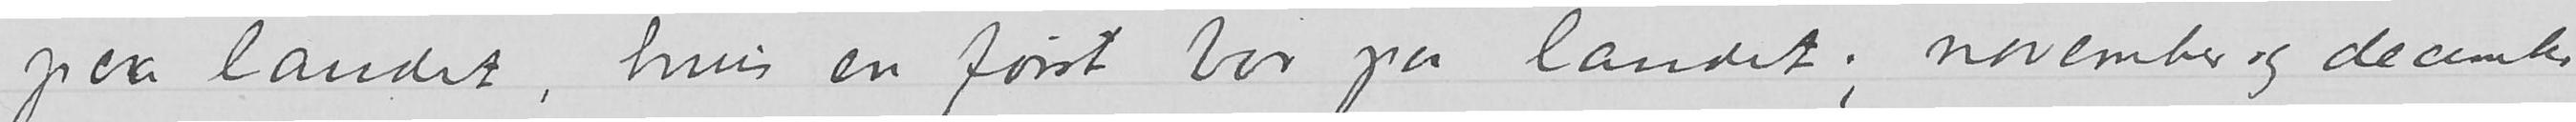paa landet, hvis en først bor paa landet; november og december
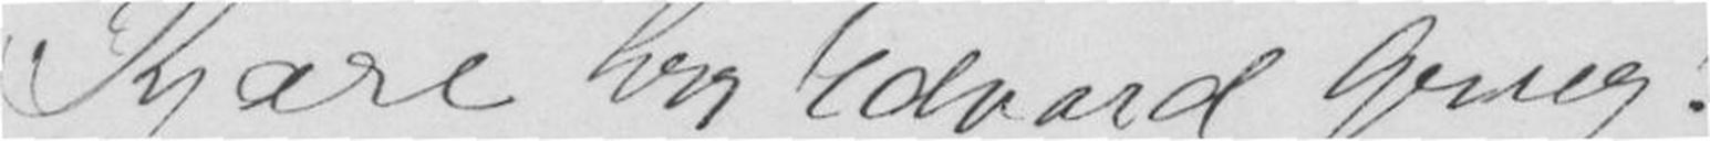Kjære hrr Edvard Grieg!
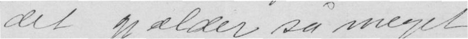det gjælder så meget
